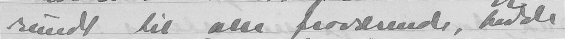rundt til alle forordrende, hadde
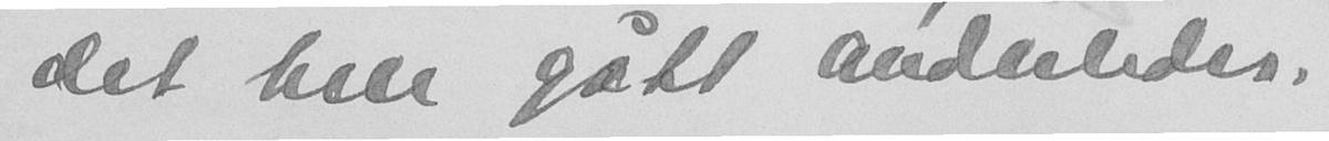det hele gått anderledes.
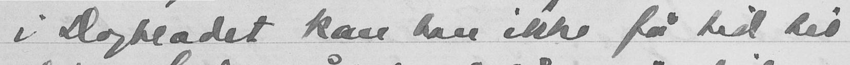i Dagbladet kan han ikke få tid til
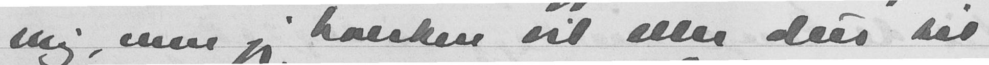mig, men jeg hverken vil eller dur til
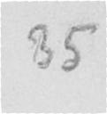35
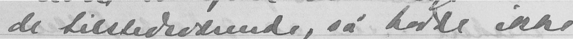de tilstedeværende, så hadde ikke
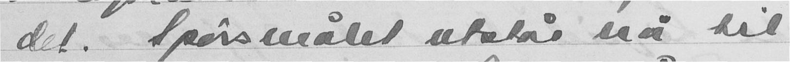det. Spørsmalet utstår nå til
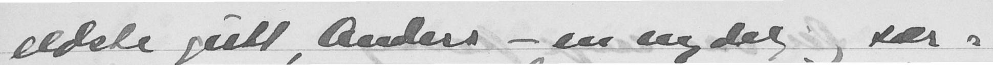eldste gutt, Anders - en nydelig og sær-
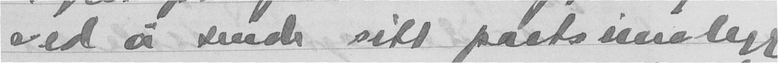ved å sende sitt postinnlegg
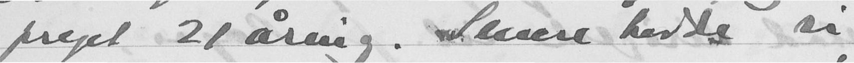preget 21 åring. Senere hadde vi
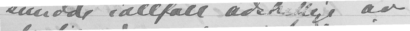snudde iallfall adskillige av
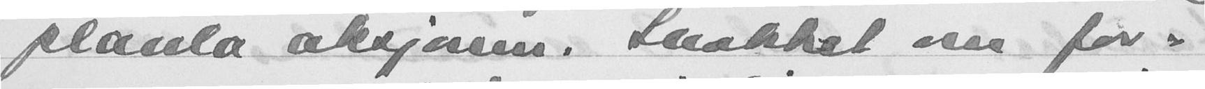planla aksjonen. Snakket om for-
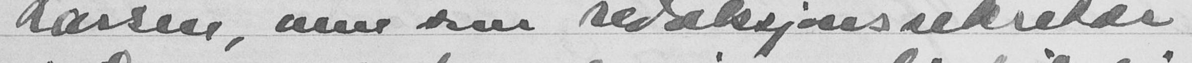Larsen, men som redaksjonssekretær
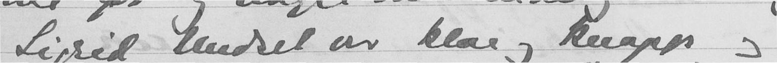Sigrid Undset var klar og knapp og
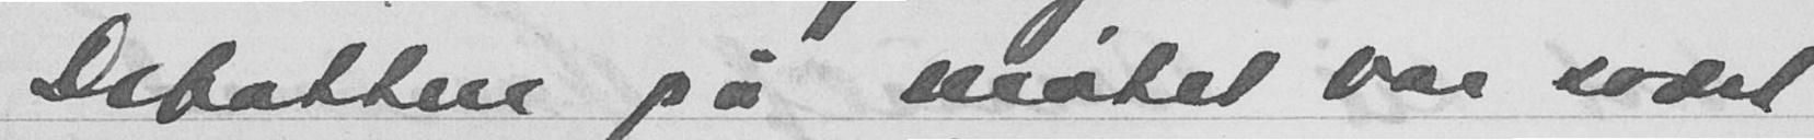Debatten på møtet var svært
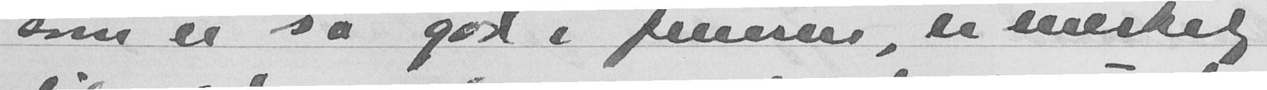som er så god i pressen, er merkelig
